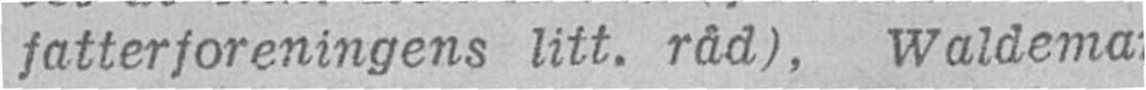fatterforeningens litt. råd) Waldemar
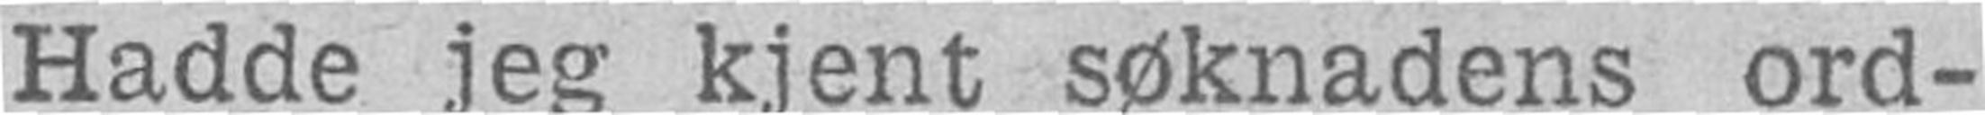Hadde jeg kjent søknadens ord-
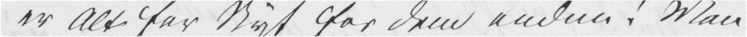er Alt for Nyt for dem endnu! Man
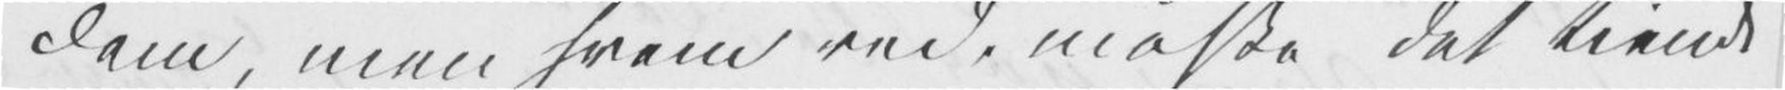dem, men hvem ved! måske det tiende
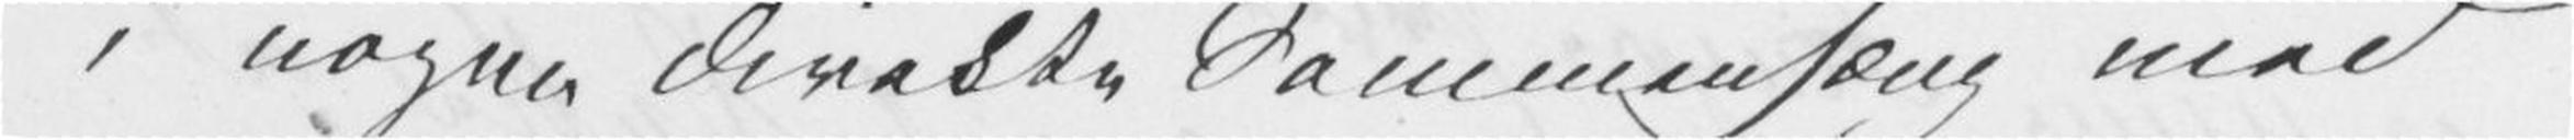i nogen direkte Sammenhæng med
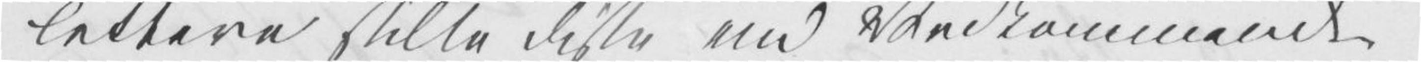lettere stille disse end Vedkommende
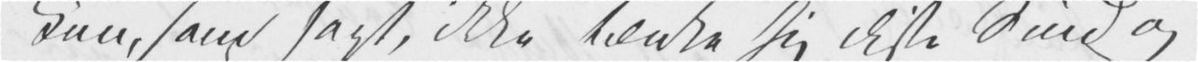kan, som sagt, ikke tænke sig disse Sind og
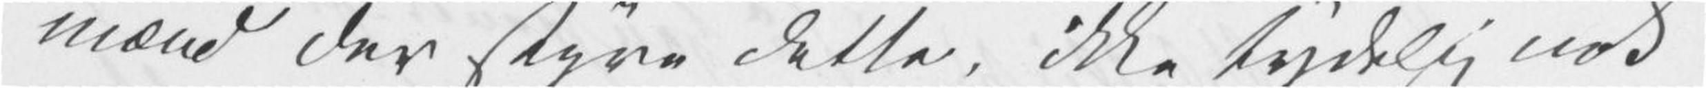mænd der styre dette, ikke tydelig nok
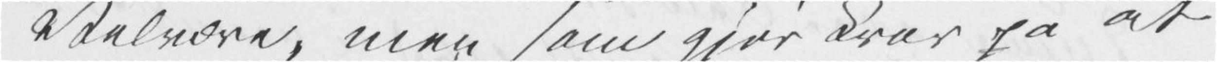Velvære, men som gjør Krav på at
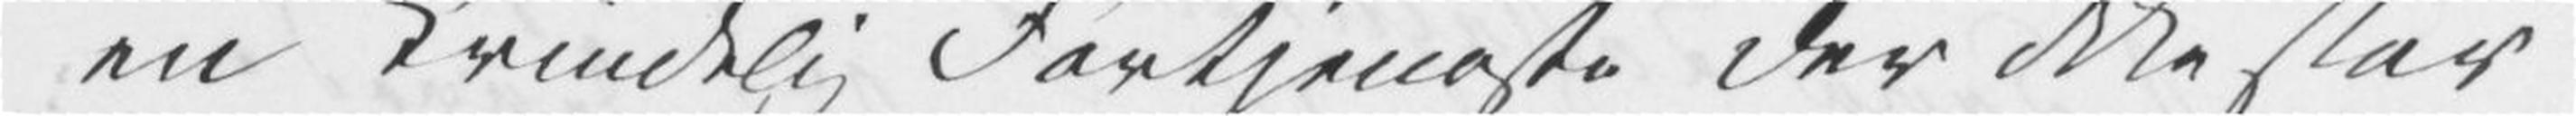en kvindelig Fortjeneste der ikke står
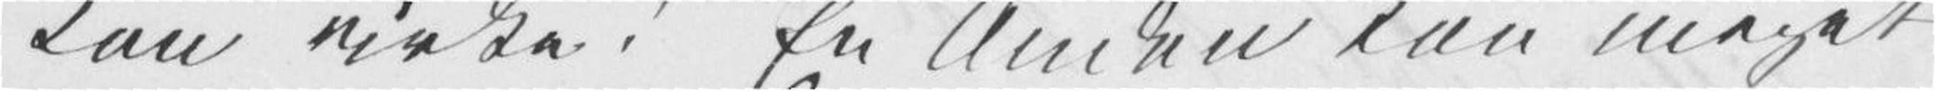kan virke! En Anden kan meget
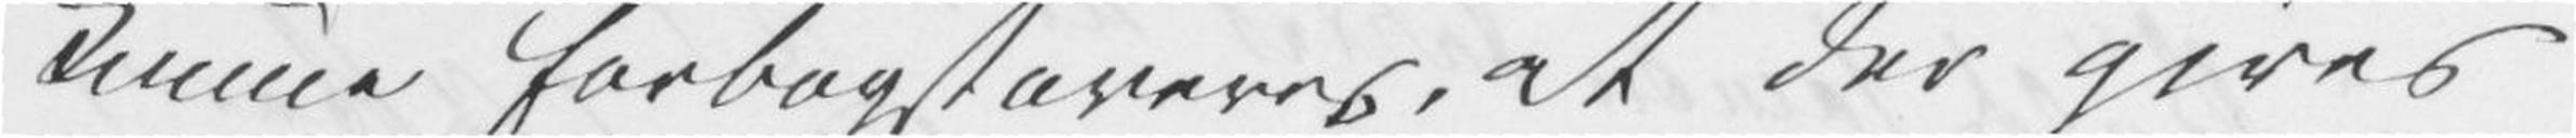kunne forbogstaveres, at der gives
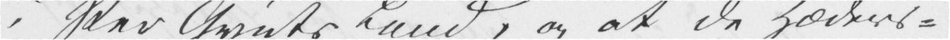i Per Gynts Land, og at de Hæders¬
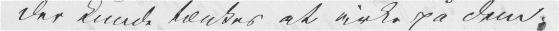der kunde tænkes at virke på dem.
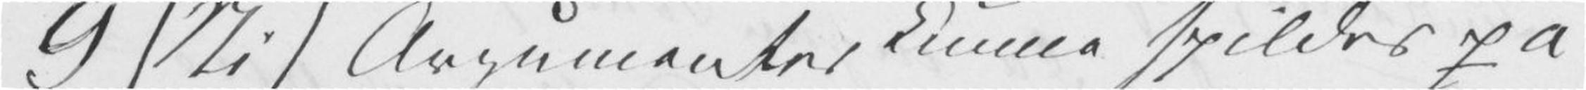9 (Ni) Argumenter kunne spildes på
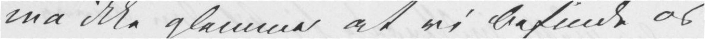må ikke glemme at vi befinde os
